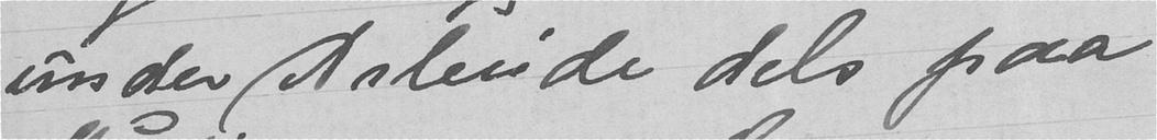under Arbeide dels paa
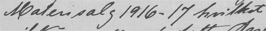Malerisalg 1916- 17 hvilket
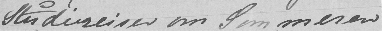Studiereiser om Sommeren
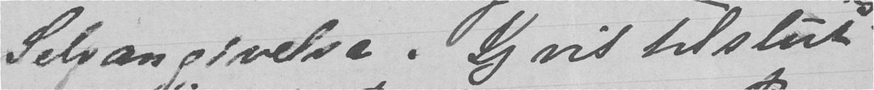Selvangivelse. Jeg vil tilstut
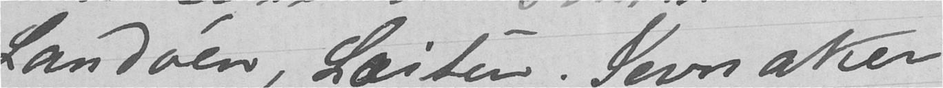Sandven, Løiten. Jevnaker
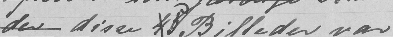der disse 45 Billeder var
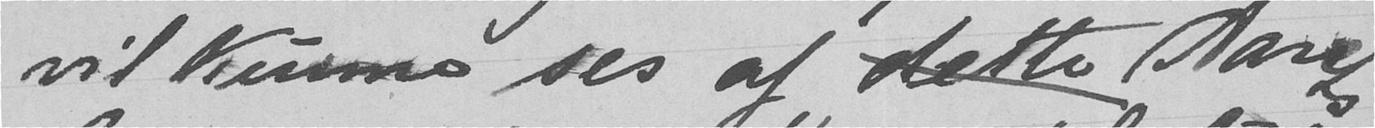vil kunne ses af dette Aarets
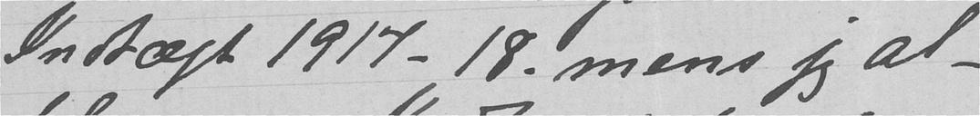Indtægt 1917-18. mens jeg al-
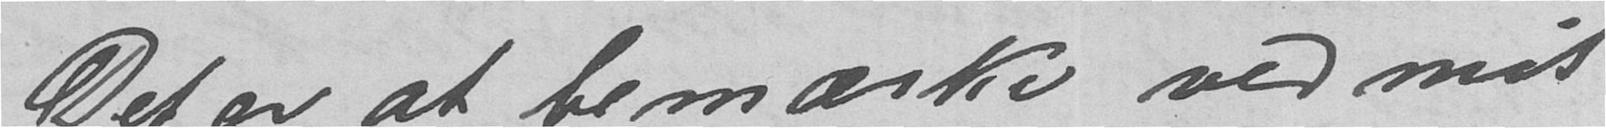Det er at bemærke ved mit
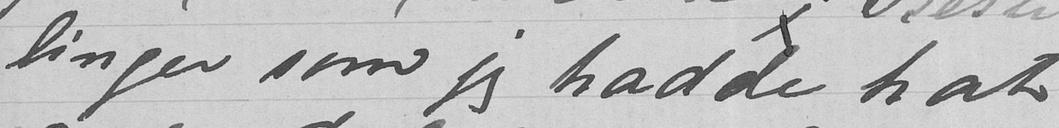linger som jeg hadde hat
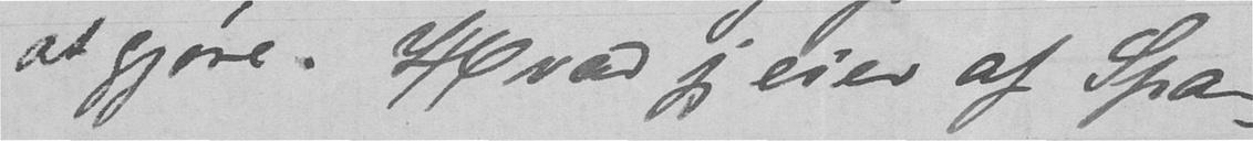at gjøre. Hvad jeg eier af Spar-
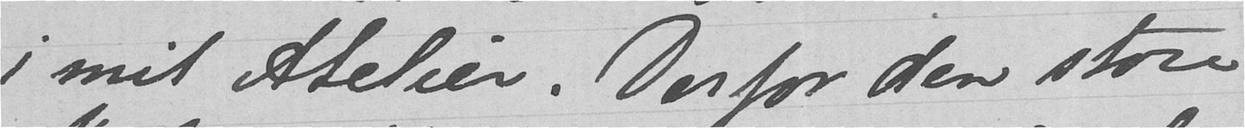i mit Atelier. Derfor den store
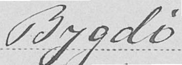Bygdø
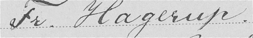Fr. Hagerup.
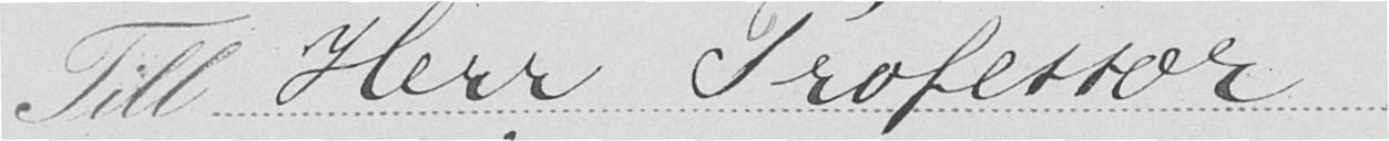Till Herr Professor
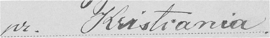pr. Kristiania
